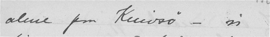alene paa Knivsø - vi
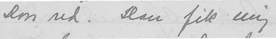Don red. Han fik mig
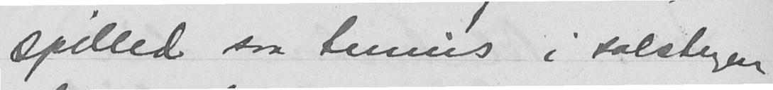spilled saa tennis i solstegen
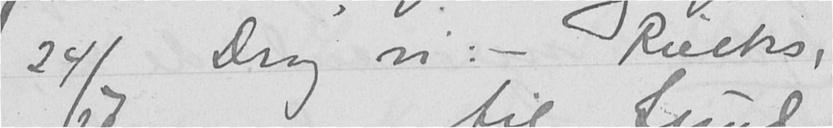24/7 Drog vi: - Riecks,
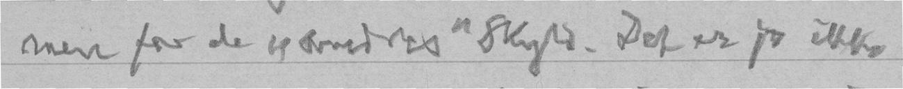men for de "andres" Skyld. Det er jo ikke
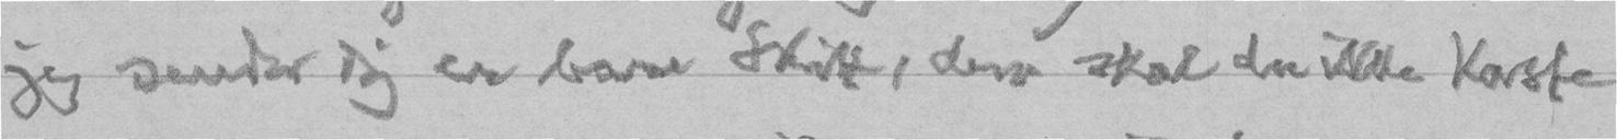jeg sender dig er bare Skitt, dem skal du ikke kaste
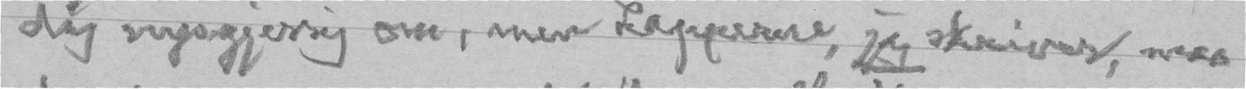dig nysgjerrig om, men Lapperne, jeg skriver, maa
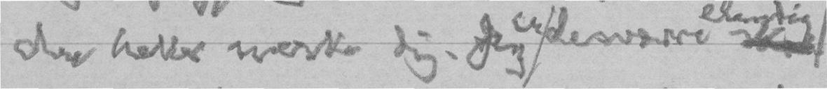du heller merke dig. Jeg er desværre skrl
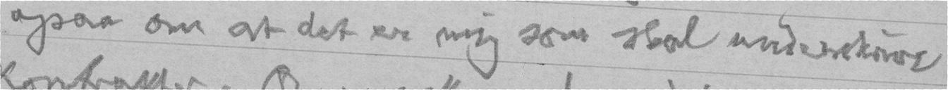ogsaa om at det er mig som skal underskrive
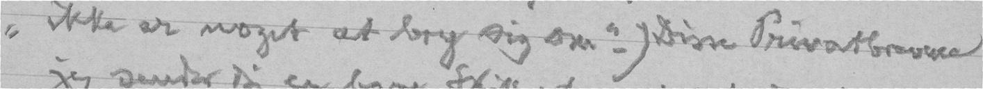"ikke er noget at bry sig om".) Disse Privatbrevene
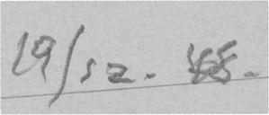19/12. 48.
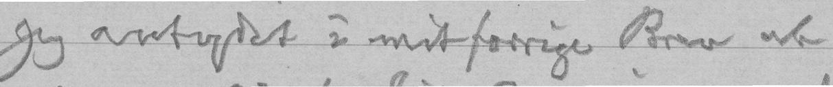Jeg antydet i mit forrige Brev at
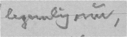legemlig nu,
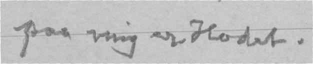paa mig er Hodet.
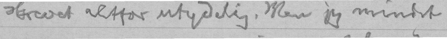skrevet altfor utydelig. Men jeg mindet
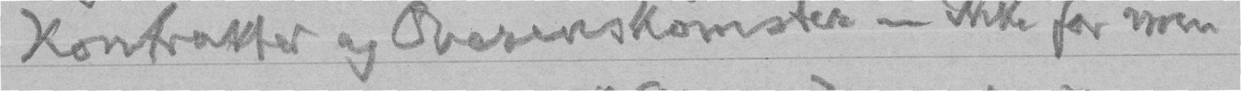Kontrakter og Overenskomster - ikke for min
